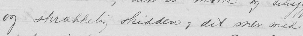og skrækkelig skidden; det sner med
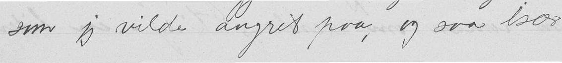som jeg vilde angret paa, og saa især
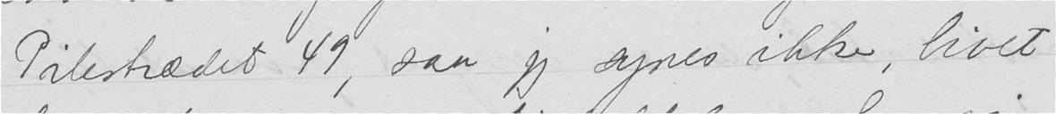Pilestrædet 49, saa jeg synes ikke, livet
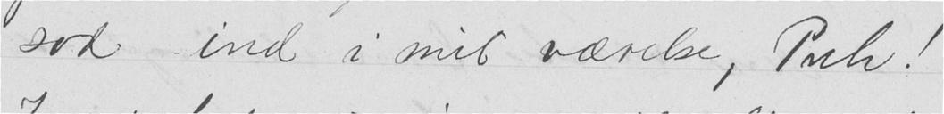sod ind i mit værelse, Puh!
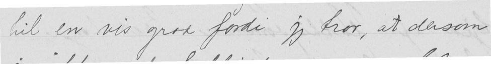til en vis grad fordi jeg tror, at dersom
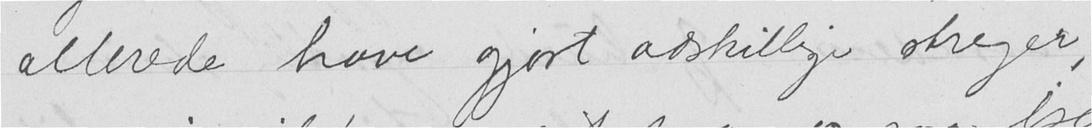allerede have gjort adskillige streger,
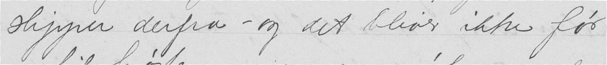slipper derfra - og det bliver ikke før
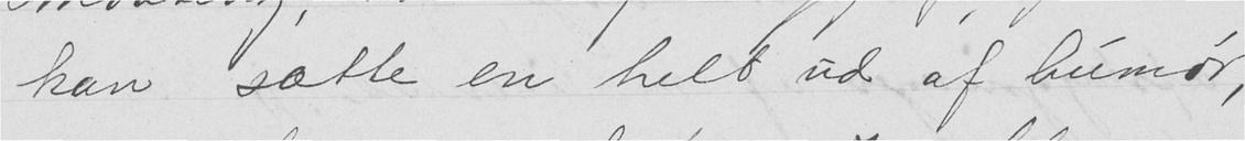kan sætte en helt ud af humør,
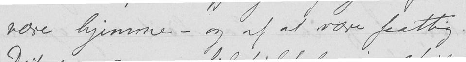være hjemme - og af at være fattig.
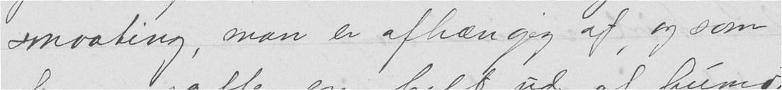smaating, man er afhængig af, og som
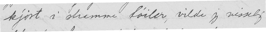kjørt i stramme tøiler, vilde jeg visselig
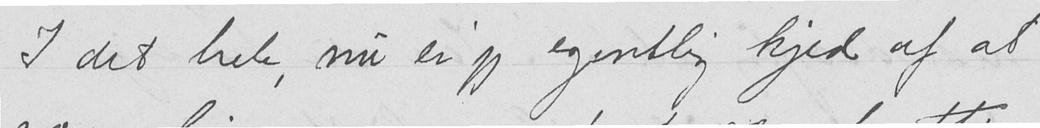I det hele, nu er jeg egentlig kjed af at
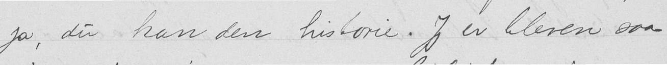ja, du kan den historie. Jeg er bleven saa
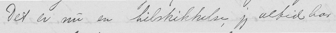Det er nu en tilskikkelse, jeg altid har
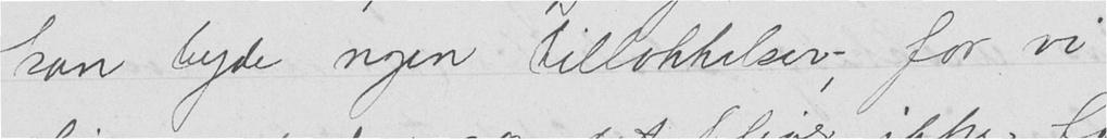kan byde nogen tillokkelser, før vi
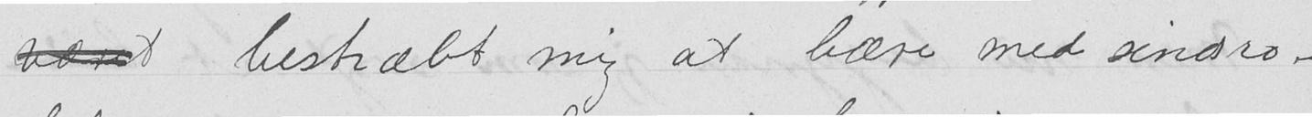vært bestræbt mig at bære med sindsro -
